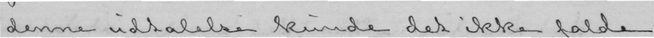denne udtalelse kunde det ikke falde
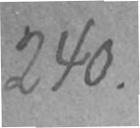240.
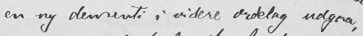en ny dementi i videre ordelag udgaa,
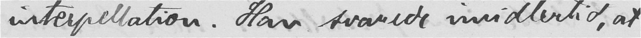interpellation. Han svarede imidlertid, at
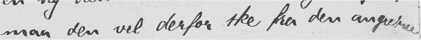maa den vel derfor ske fra den angrebne
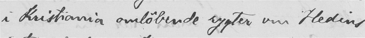i Kristiania omløbende rygter om Hedins
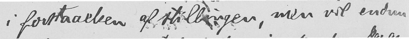i forstaaelsen af stillingen, men vil endnu
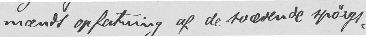mænds opfatning af de svævende spørgs-
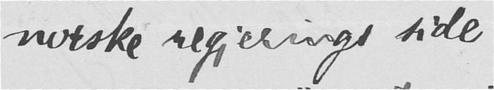norske regjerings side.
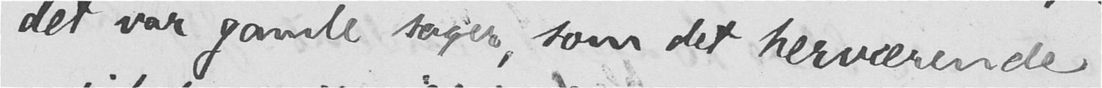det var gamle sager, som det herværende
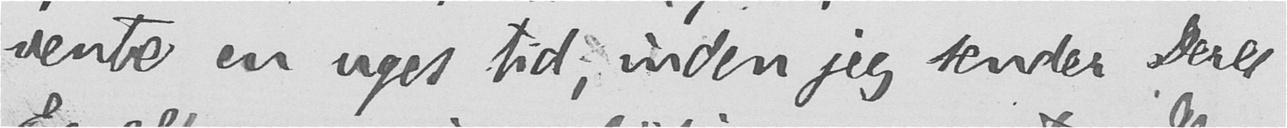vente en uges tid, inden jeg sender Deres
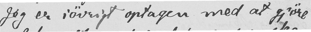Jeg er iøvrigt optagen med at gjøre
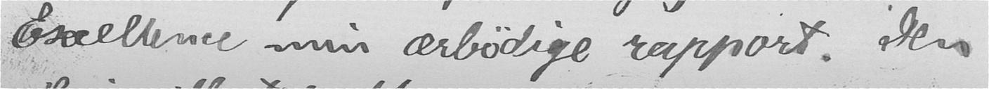Excellence min ærbødige rapport. Den
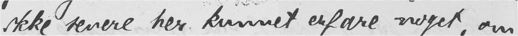ikke senere her kunnet erfare noget, om
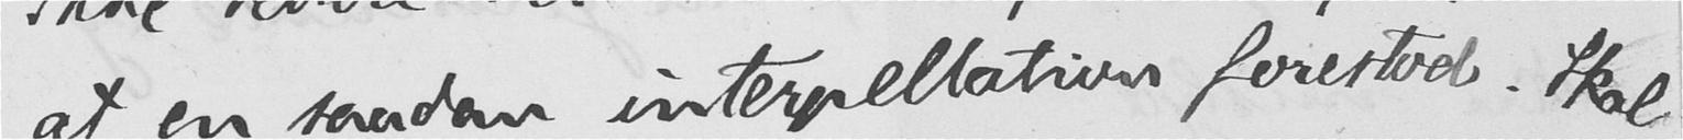at en saadan interpellation forestod. Skal
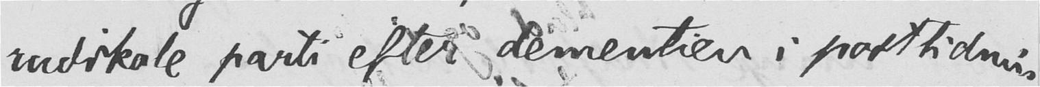radikale parti efter dementien i posttidnin-
